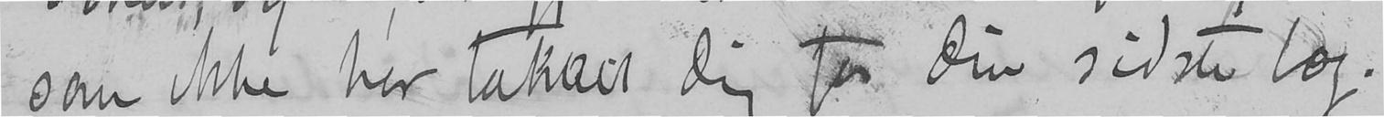som ikke har takket dig for din sidste bog.
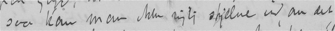saa kan man ikke rigtig skjelne ud om det,
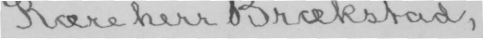Kære herr Brækstad,
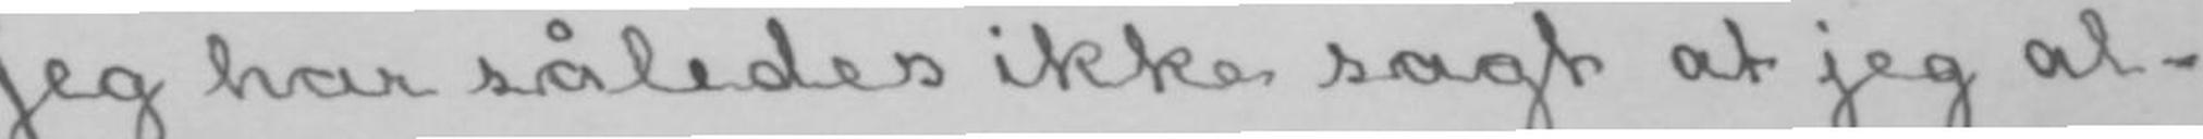Jeg har således ikke sagt at jeg al-
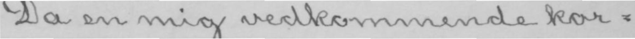Da en mig vedkommende kor-
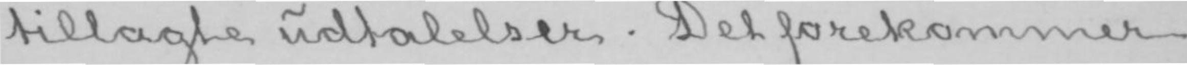tillagte udtalelser. Det forekommer
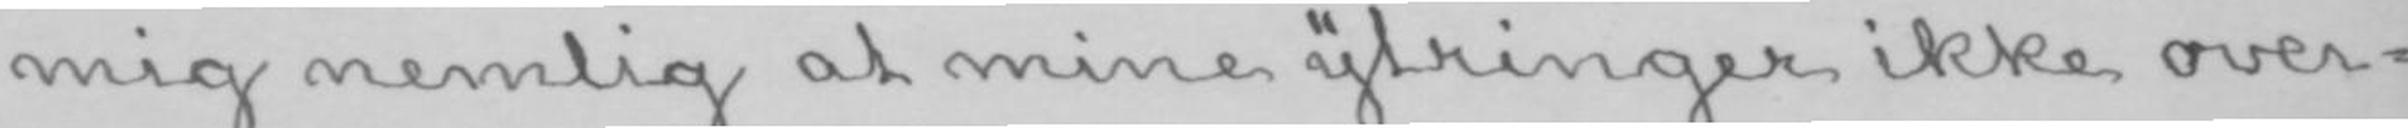mig nemlig at mine ytringer ikke over-
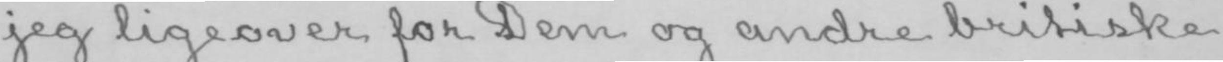jeg ligeover for Dem og andre britiske
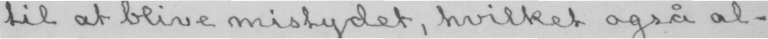til at blive mistydet, hvilket også al-
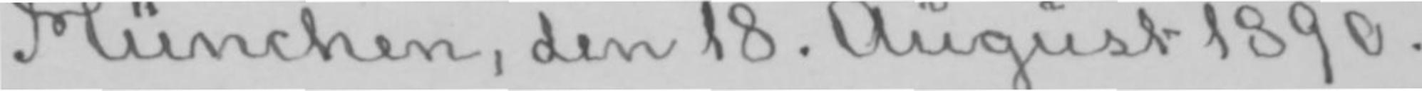München, den 18. August 1890.
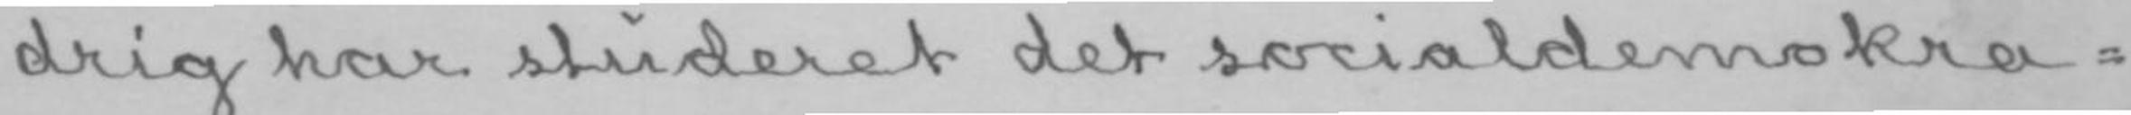drig har studeret det socialdemokra-
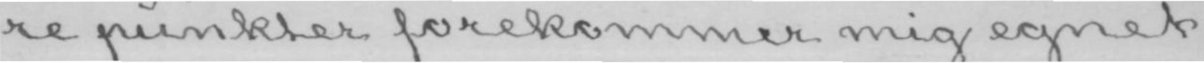re punkter forekommer mig egnet
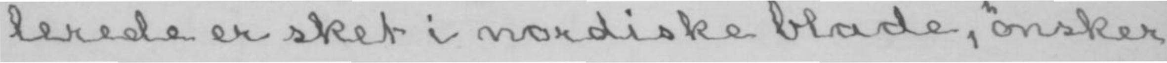lerede er sket i nordiske blade, ønsker
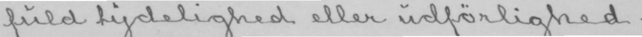fuld tydelighed eller udførlighed.
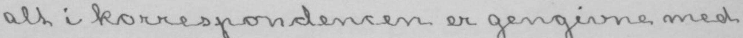alt i korrespondencen er gengivne med
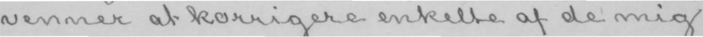venner at korrigere enkelte af de mig
